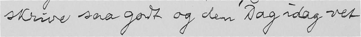skrive saa godt og den Dag idag vet
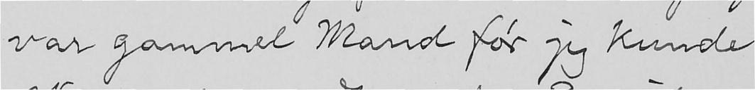var gammel Mand før jeg kunde
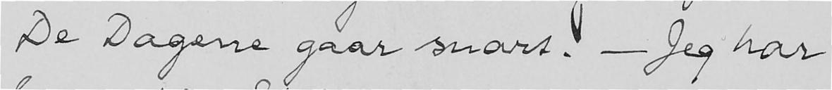De Dagene gaar snart. - Jeg har
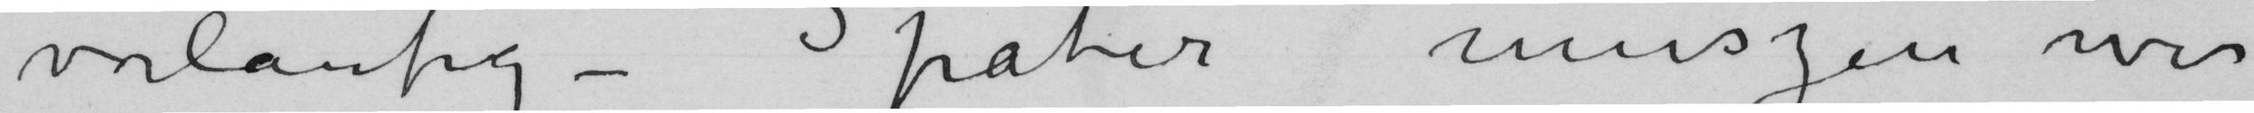vorlaufig – Später muszen wir
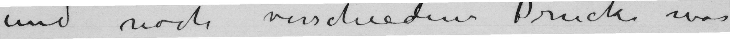und noch verschiedene Drucke was
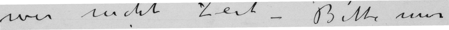wir nicht Zeit – Bitte mir
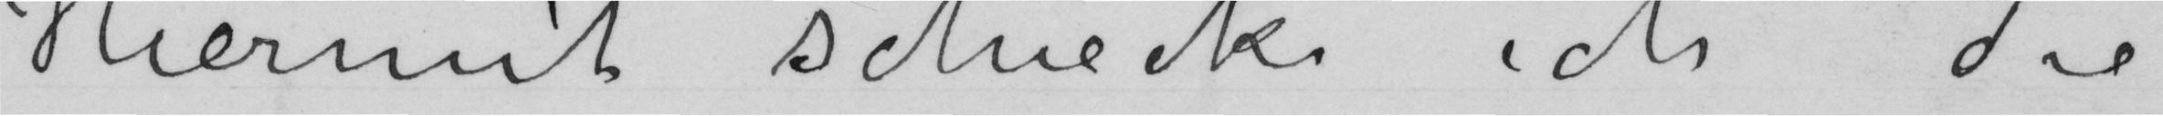Hiermit schiecke ich die
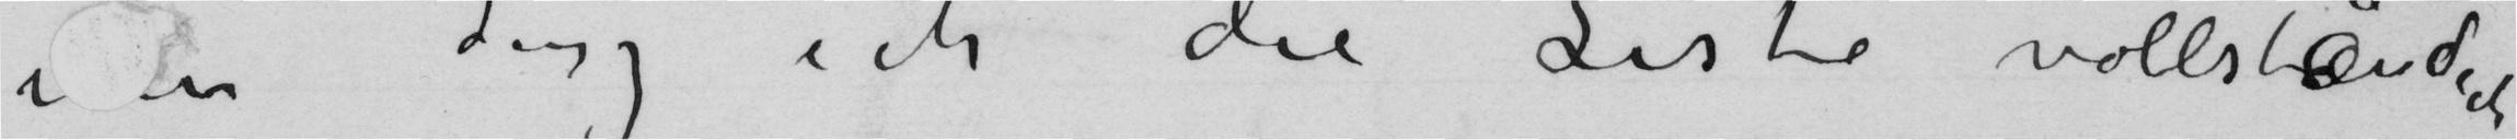dasz ich die Liste vollständich
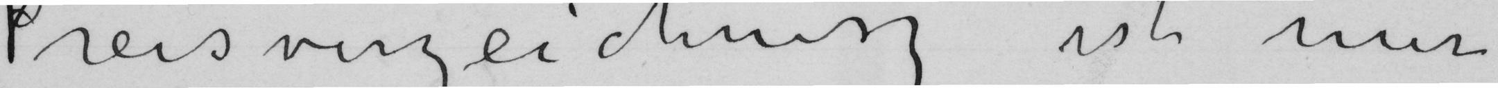Preisverzeichnisz ist nur
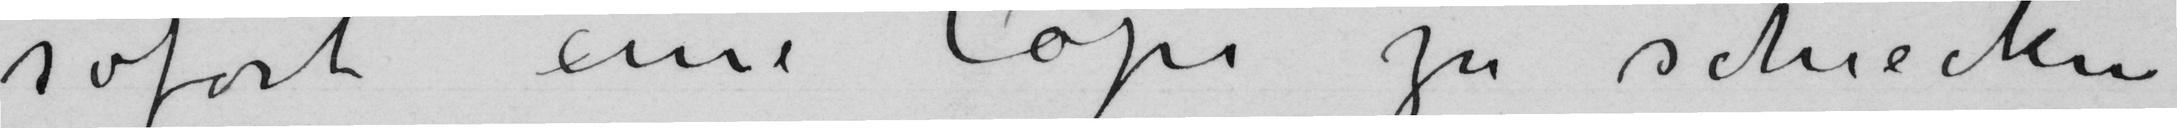sofort eine Copi zu schiecken
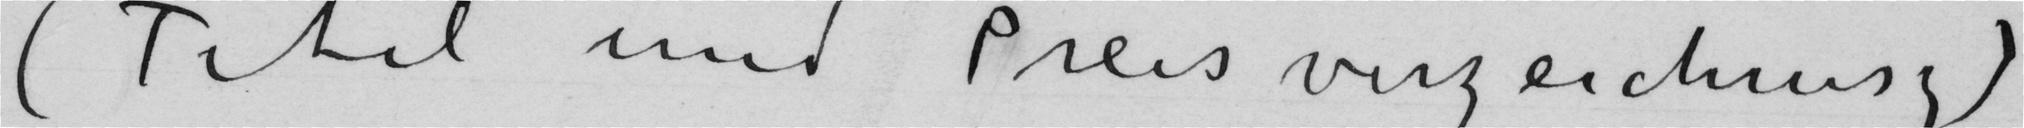(Titel und Preisverzeichnisz)
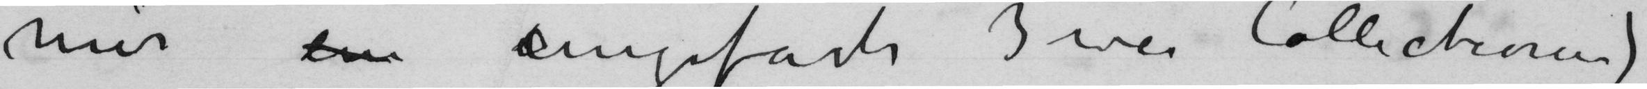mir ein angefach zwei Collectionen)
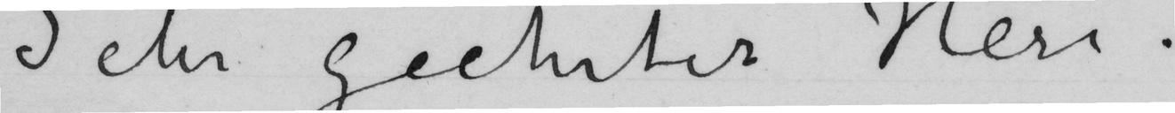Sehr geehrter Herr.
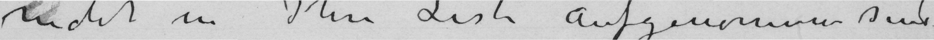nicht in Ihre Liste aufgenommen sind
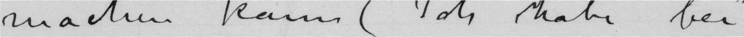machen kann (Ich habe bei
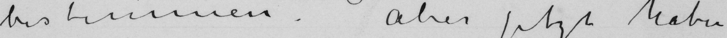bestimmen. Aber jetzt haben
